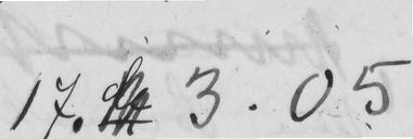17. 3.05
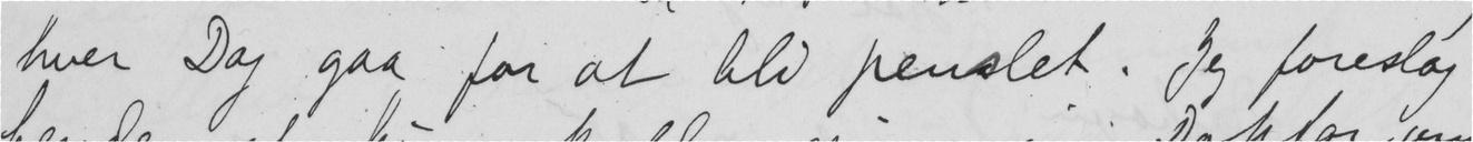hver Dag gaa for at bli penslet. Jeg foreslog
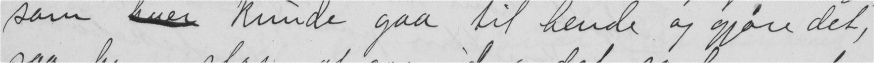som  kunde gaa til hende og gjøre det,
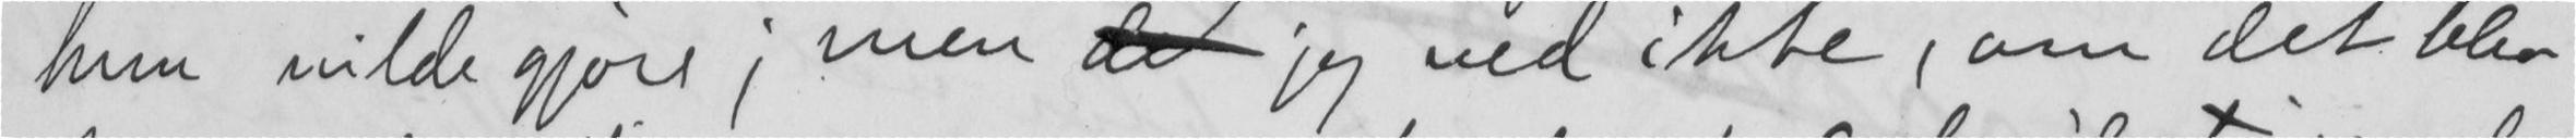hun vilde gjøre; men  jeg ved ikke, om det blev
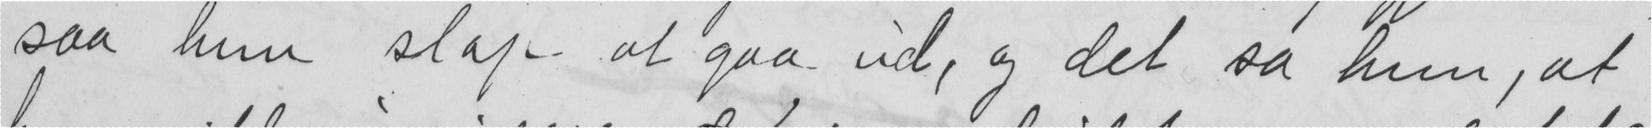saa hun slap at gaa ud, og det sa hun, at
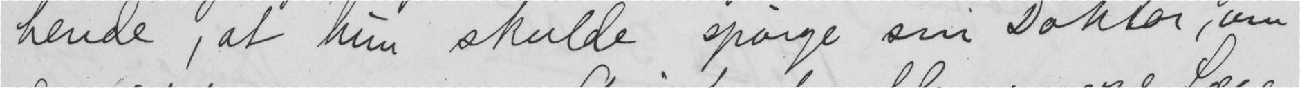hende, at hun skulde spørge sin Doktor, om
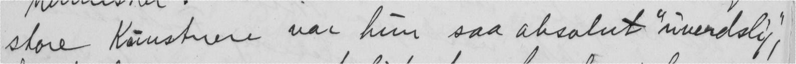store Kunstnere var hun saa absolut "uverdslig",
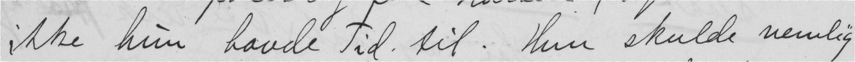ikke hun havde Tid til. Hun skulde nemlig
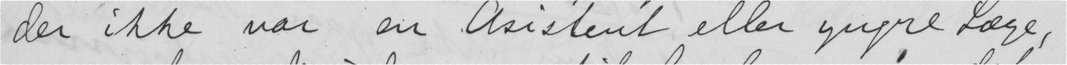der ikke var en Asistent eller yngre Læge,
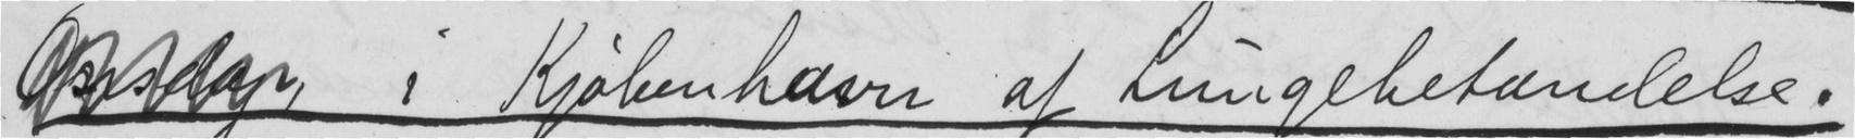i Kjøbenhavn af Lungebetændelse.
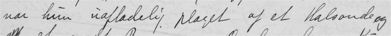var hun uafladelig plaget af et Halsonde og
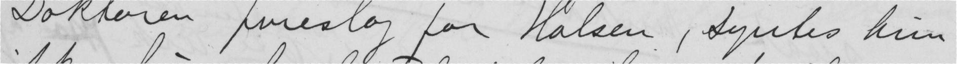Doktoren foreslog for Halsen, syntes hun
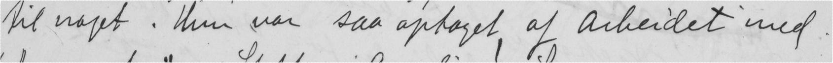til noget. Hun var saa optaget af Arbeidet med
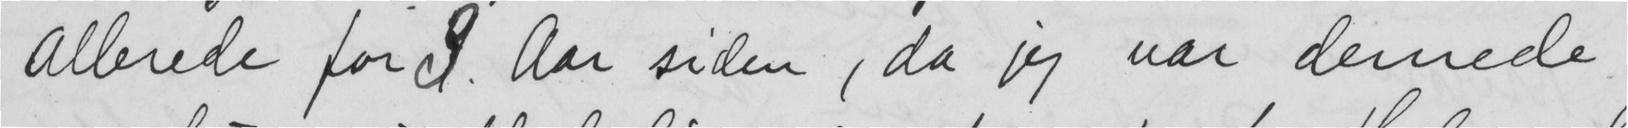Allerede for 3 Aar siden, da jeg var dernede
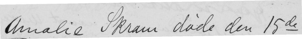Amalie Skram døde den 15de
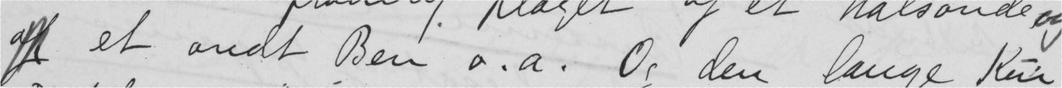et ondt Ben o.a. Og den lange Kur
